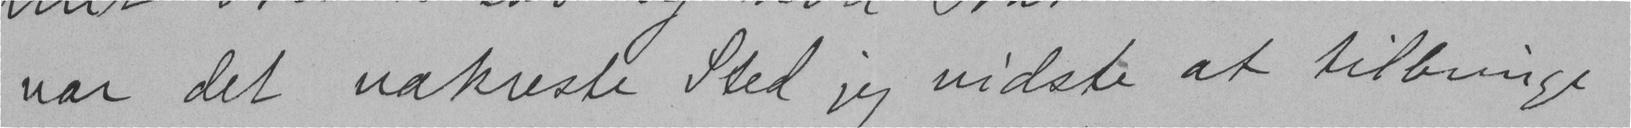var det vakreste Sted jeg vidste at tilbringe
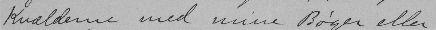Kvælderne med mine Bøger eller
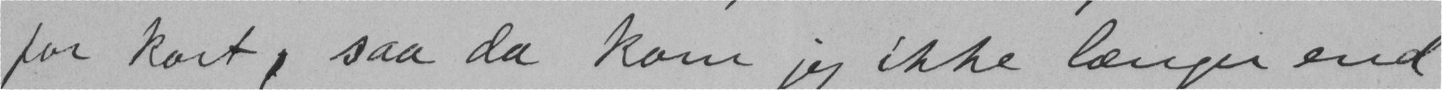for kort, saa da kom jeg ikke længer end
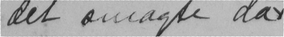det smagte da
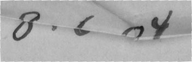8.6.04
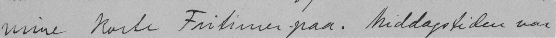mine korte Fritimer paa. Middagstiden var
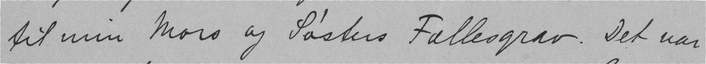til min Mors og Søsters Fællesgrav. Det var
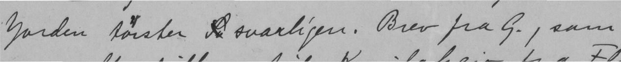Jorden tørster  svarligen. Brev fra G., som
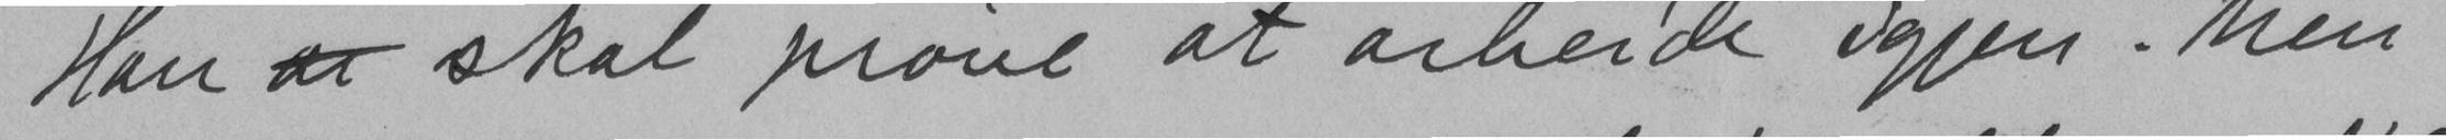Han  skal prøve at arbeide igjen. Men
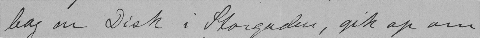bag en Disk i Storgaden, gik op om
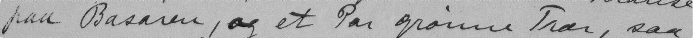paa Basaren, og et Par grønne Trær, saa
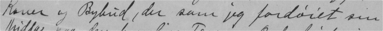Koner og Bybud, der som jeg fordøiet sin
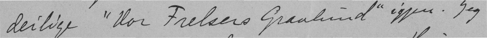deilige "Vor Frelsers Gravlund" igjen. Jeg
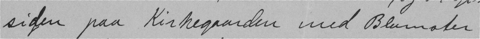siden paa Kirkegaarden med Blomster
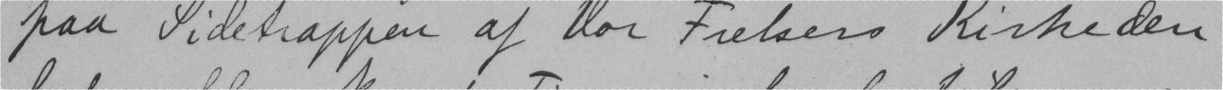paa Sidetrappen af Vor Frelsers Kirke den
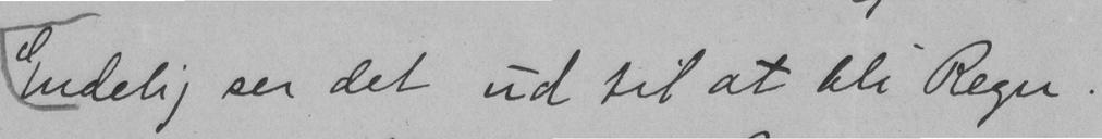Endelig ser det ud til at bli Regn.
